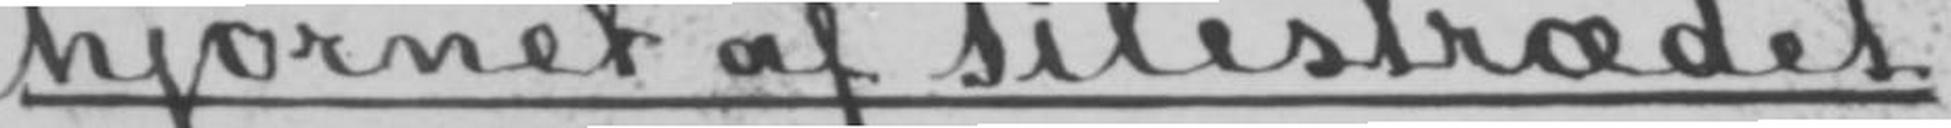hjørnet af Pilestrædet
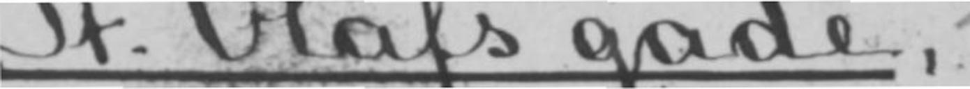St. Olafs gade,
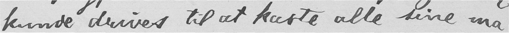kunde drives til at kaste alle sine ma-
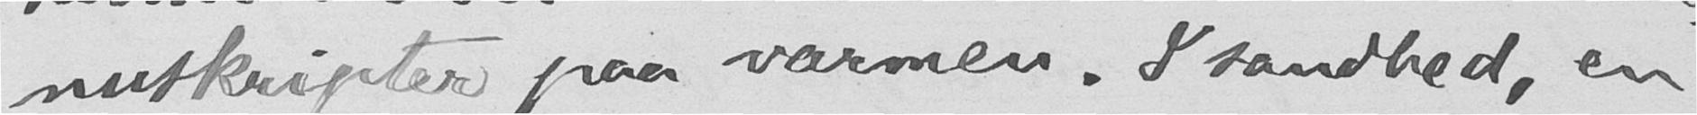nuskripter paa varmen. I sandhed, en
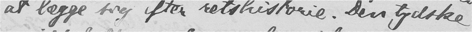at lægge sig efter retshistorie. Den tydske
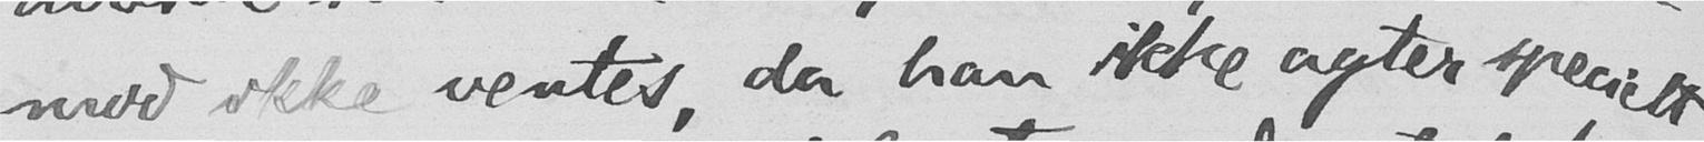mod ikke ventes, da han ikke agter specielt
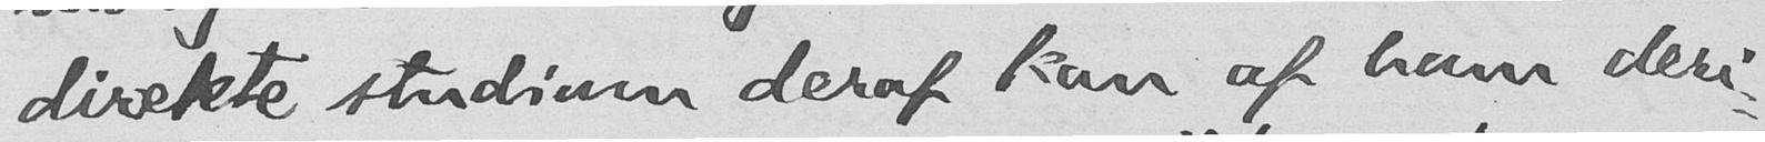direkte studium deraf kan af ham deri-
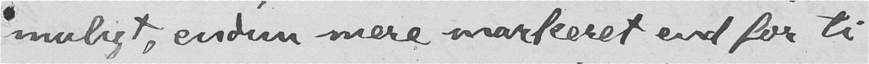muligt, endnu mere markeret end for ti
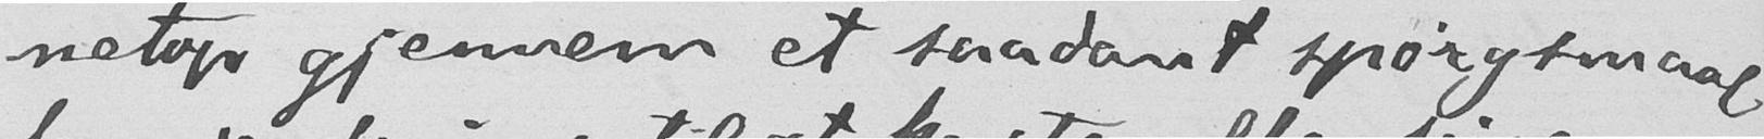netop gjennem et saadant spørgsmaal
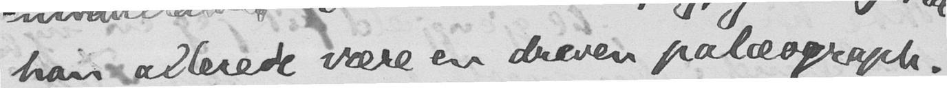han allerede være en dreven palæograph.
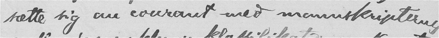sætte sig au courant med manuskripternes
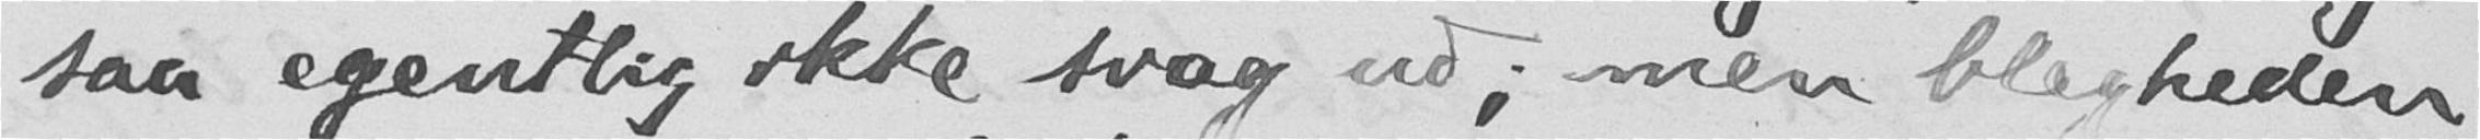saa egentlig ikke svag ud; men blegheden
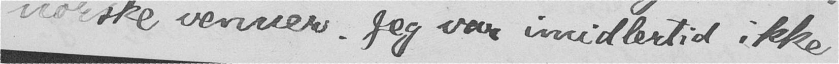norske venner. Jeg var imidlertid ikke
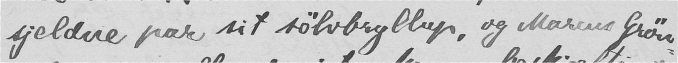sjeldne par sit sølvbryllup, og Marcus Grøn-
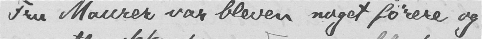Fru Maurer var bleven noget førere og
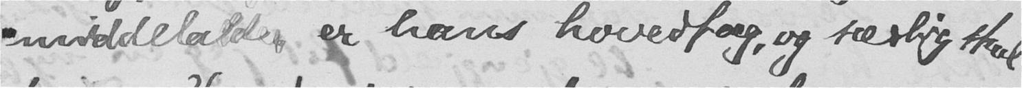middelalder er hans hovedfag, og særlig skal
